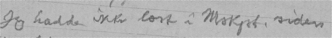Jeg hadde ikke læst i Mskpt. siden
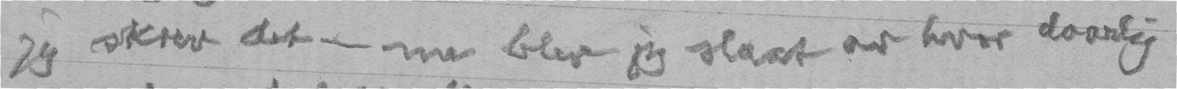jeg skrev det - nu blev jeg slaat av hvor daarlig
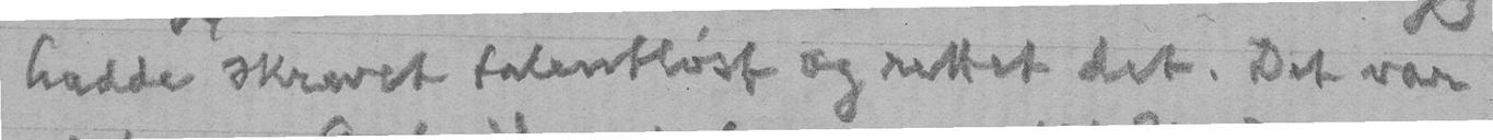hadde skrevet talentløst og rettet det. Det var
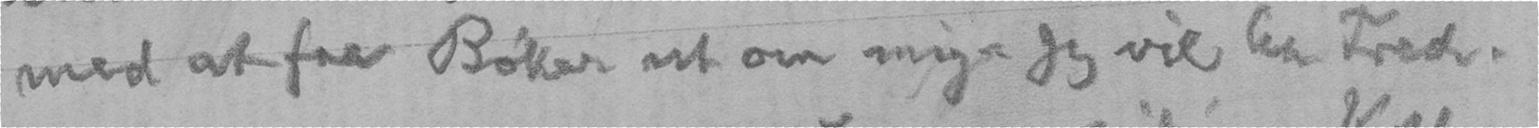med at faa Bøker ut om mig. Jeg vil ha Fred.
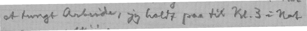et tungt Arbeide, jeg holdt paa til Kl. 3 i Nat
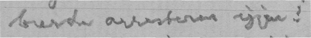burde arresteres igjen!
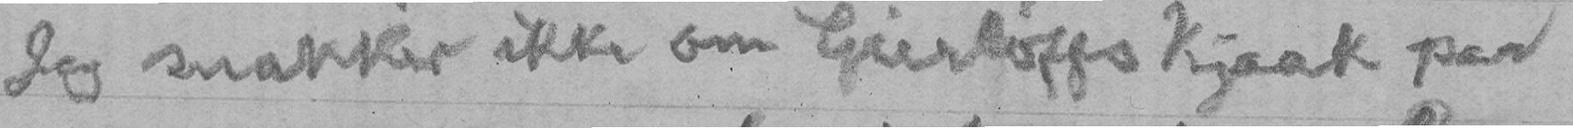Jeg snakker ikke om Gierløffs Kjaak paa
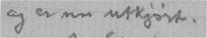og er nu utkjørt.
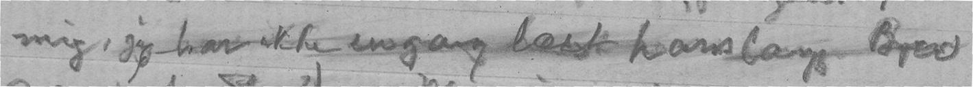mig, jeg har ikke engang læst hans lange Brev
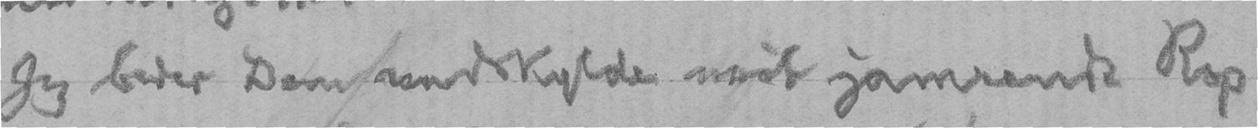Jeg beder Dem undskylde mit jamrende Rop
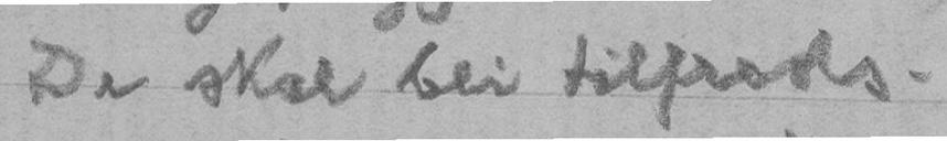De skal bli tilfræds.
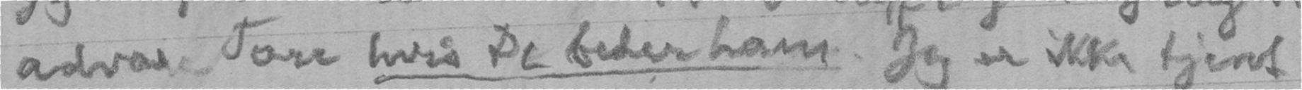advare Tore hvis De beder ham. Jeg er ikke tjent
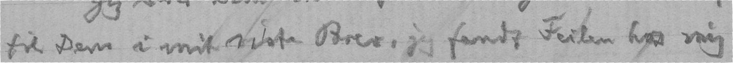til Dem i mit siste Brev, jeg fandt Feilen hos mig
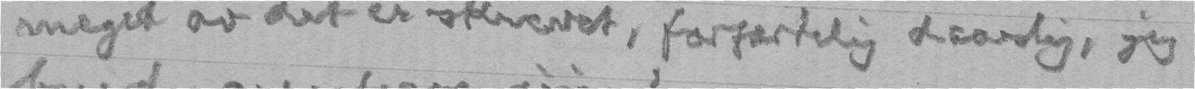meget av det er skrevet, forfærdelig daarlig, jeg
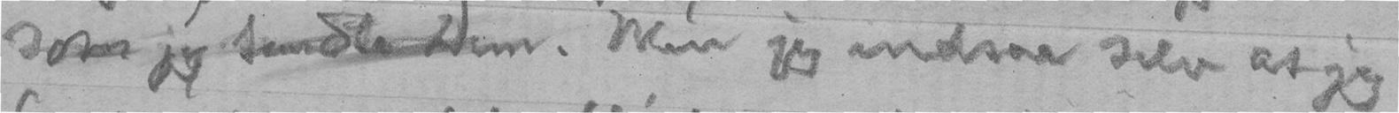som jeg sendte Dem. Men jeg indsaa selv at jeg
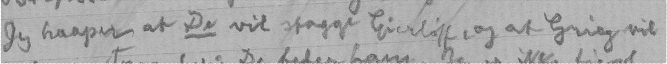Jeg haaper at De vil stagge Gierløff, og at Grieg vil
